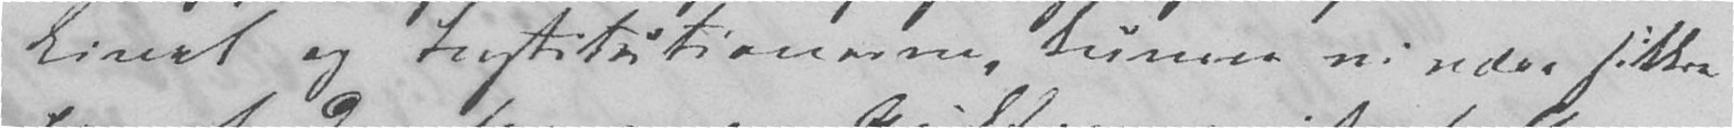Livet og Institutionerne, kunne vi være sikre
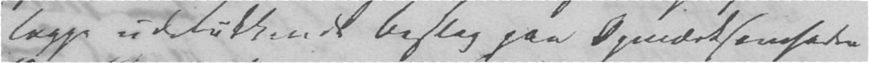lægge udelukkende Beslag paa Opmærksomheden
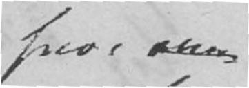som hvor
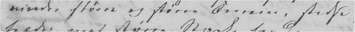vinder større og større Terrain, stedse
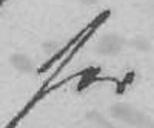for
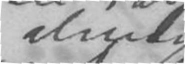almene
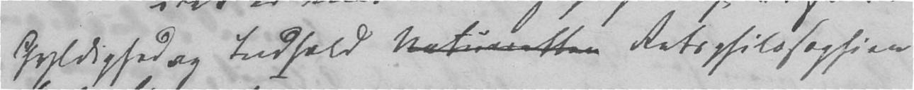Gyldighed og Indhold Naturretten Retsphilosophien
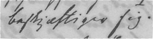beskjæftiger sig.
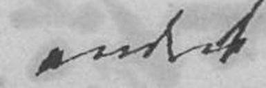andet
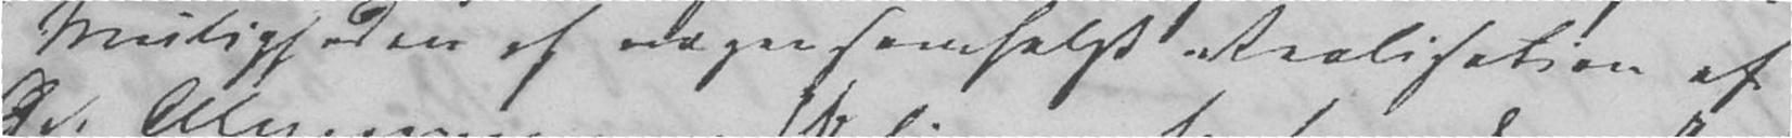Muligheden af nogensomhelst Realisation af
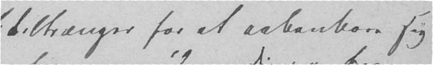tiltrænger for at aabenbare sig
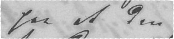paa at den
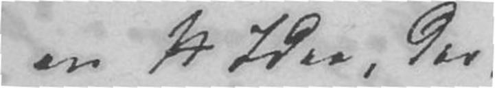en Idee, der
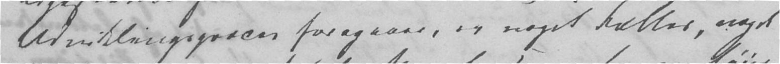Udviklingsproces foregaaer, er noget Fælles, noget
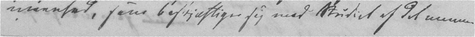meenhed, som beskjæftiger sig med Studiet af det menne-
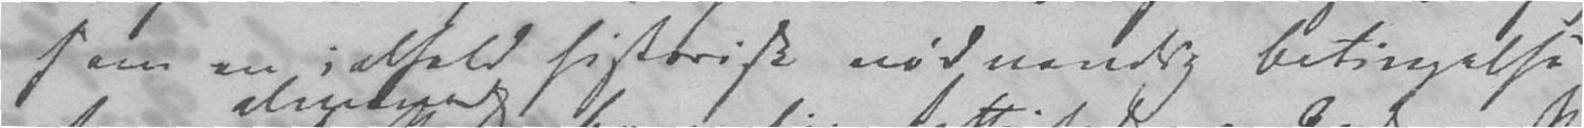som en ialfald historisk nødvendig Betingelse
